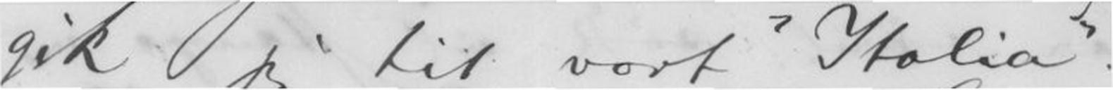gik jeg til vort "Italia".
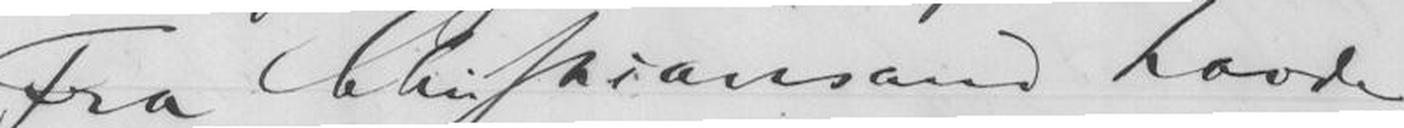Fra Christiansand havde
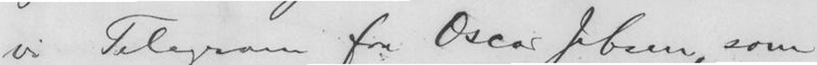vi Telegram fra Oscar Jebsen, som
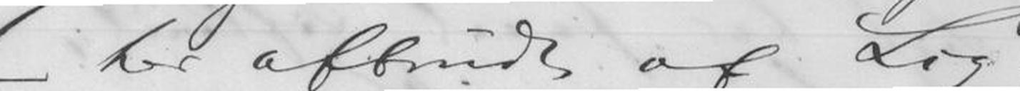(- her afbrudt af Lig-
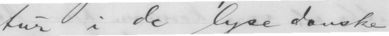tur i de lyse danske
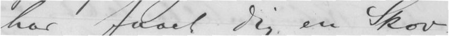har faaet Dig en Skov-
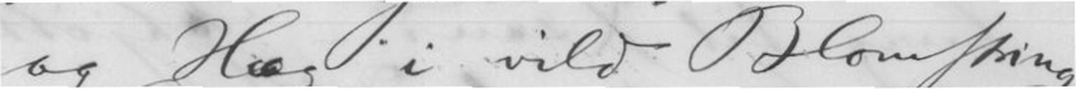og Hæg i vild Blomstring
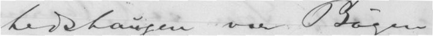hedshaugen var Bøgen
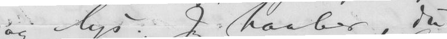og lys. Jeg haaber, Du
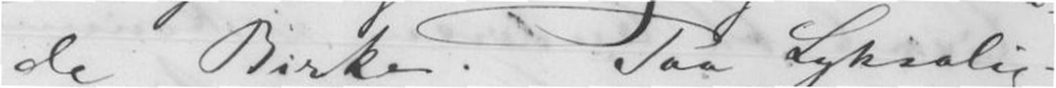de Birke. Paa Lyksalig-
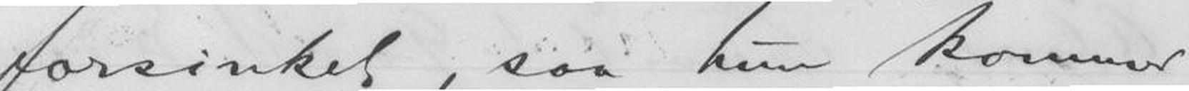forsinket, saa hun kommer
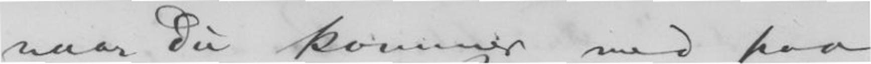naar Du kommer med paa
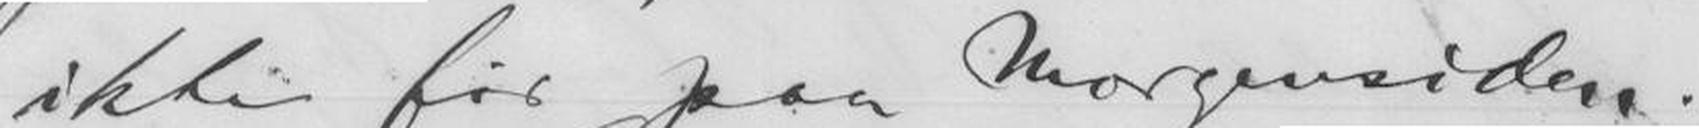ikke før paa Morgensiden.
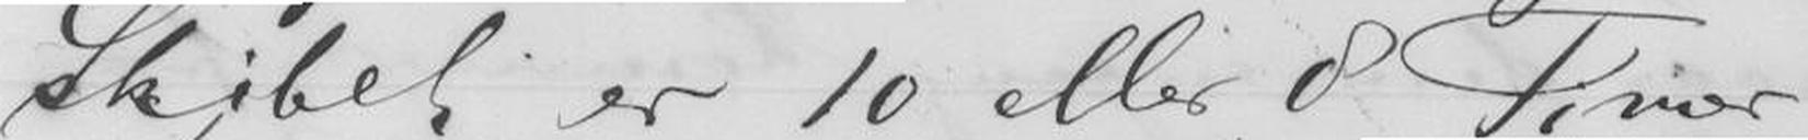Skibet er 10 eller 8 Timer
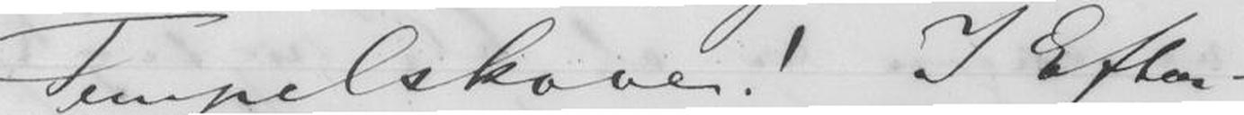Tempelskove! I Efter-
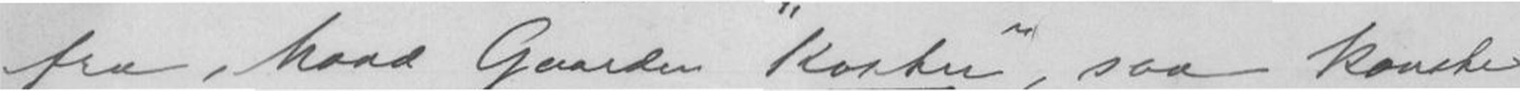fra, hvad Gaarden "Koster", saa kanske
